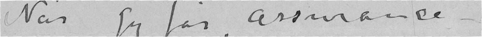Når jeg får assurance-
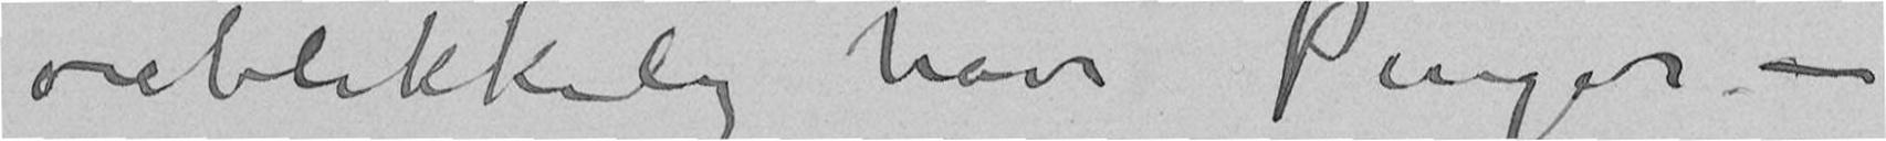øieblikkelig have Penger –
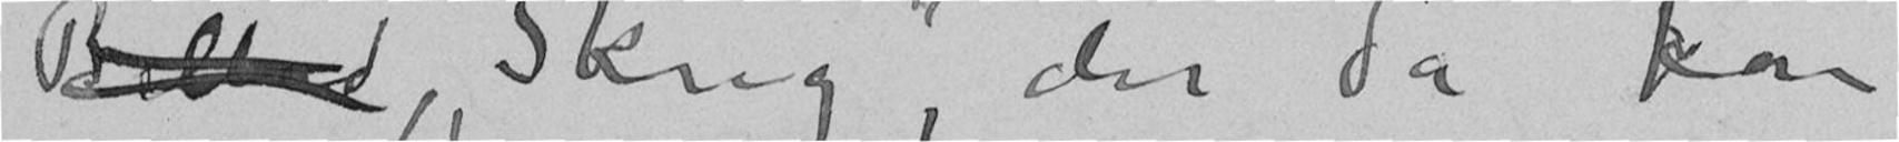Billed «Skrig», der da kan
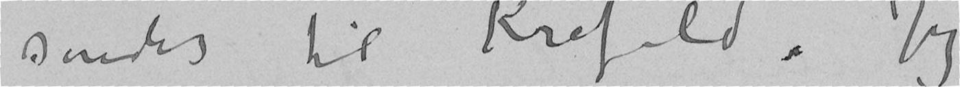sendes til Krefeld. Jeg
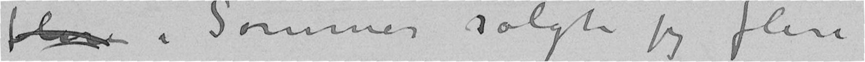flere i Sommer solgte jeg flere
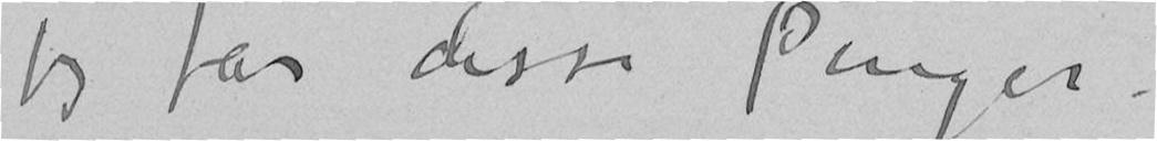jeg får disse Penger.
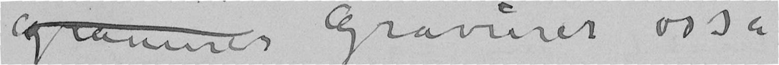gravurer Gravürer osså
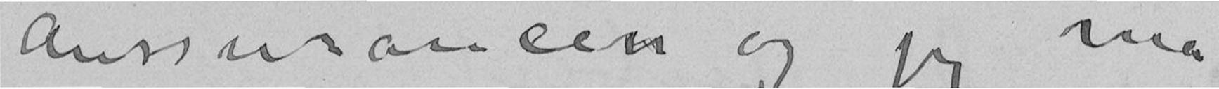Assurancen og jeg må
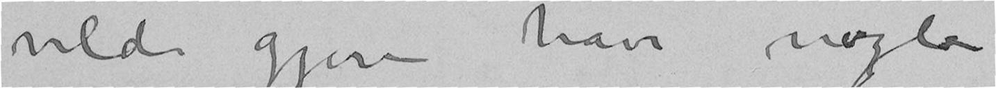vilde gjerne have nogle
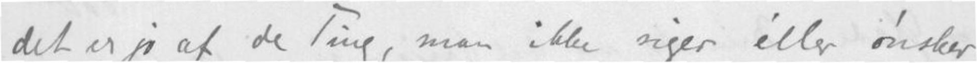det er jo af de Ting, man ikke siger eller Ønsker
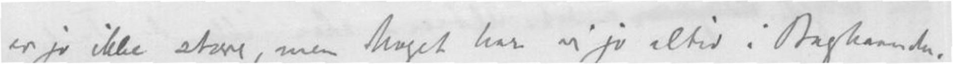er jo ikke store, men noget har vi jo altid i Baghaanden
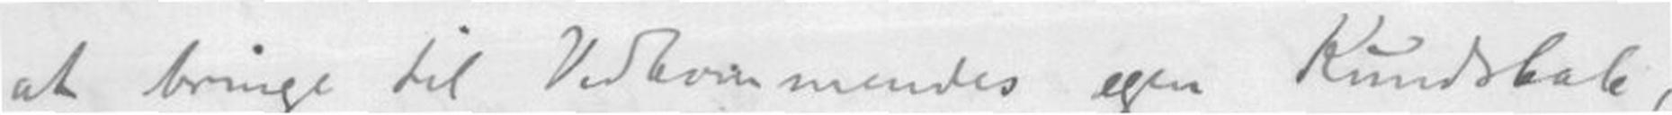at bringe til Vedkommendes egen Kundstale,
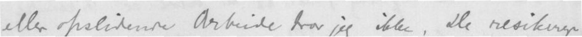eller opslitende Arbeide tror jeg ikke, De resikerer
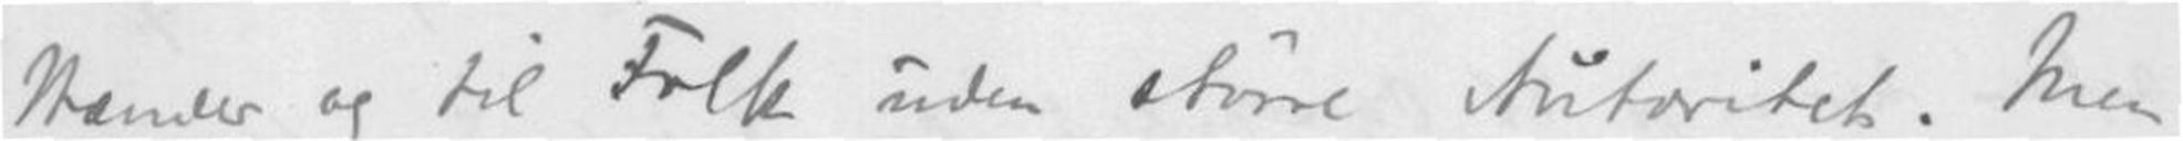Hænder og til Folk uden større Autoritet. Men
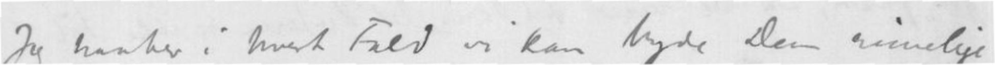Jeg haaber i hvert Fald vi kan bryde Dem rimelige
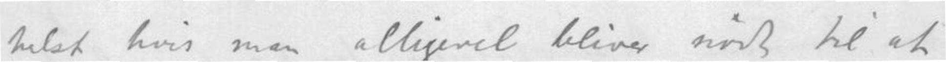helst hvis man alligevel blive nødt til at
